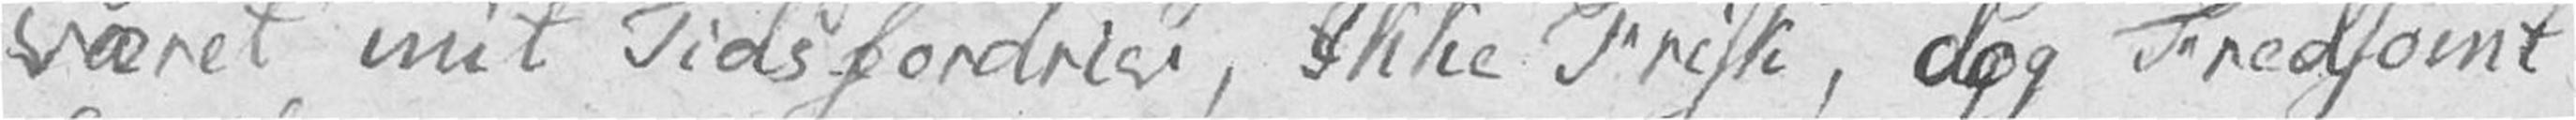været mit Tidsfordriv, Ikke Frisk, dog Fredsomt
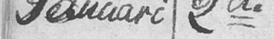Januari 2de
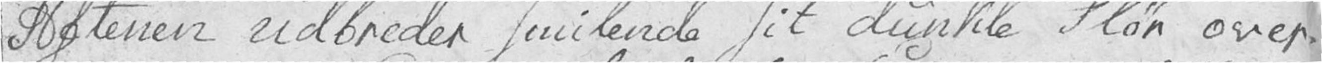Aftenen udbreder smilende sit dunkle Slør over
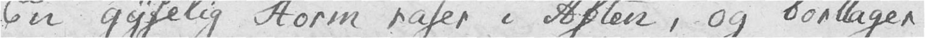En gyselig Storm raser i Aften, og borttager
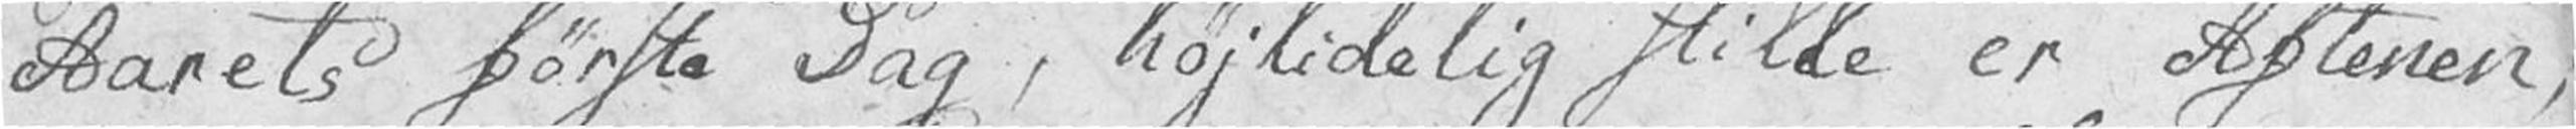Aarets første Dag, højtidelig stilde er Aftenen,
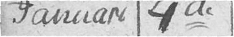Januari 4de
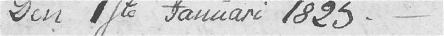Den 1ste Januari 1825. –
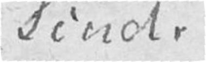Sind.
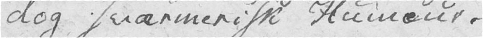dog sværmerisk Humeur.
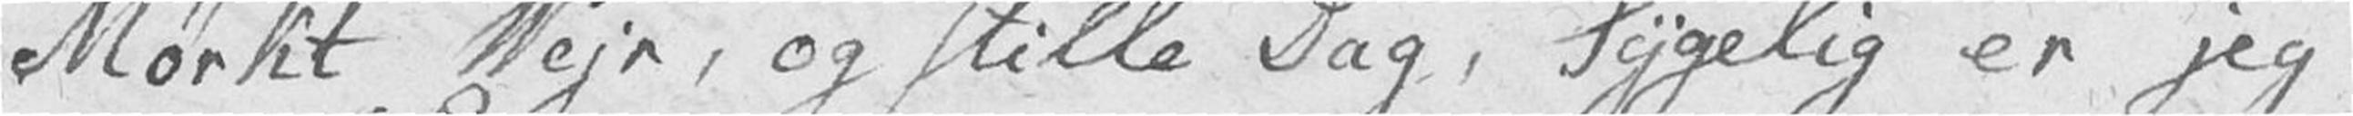Mørkt Vejr, og stille Dag, Sygelig er jeg,
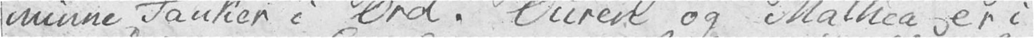minne Tanker i Ord. Ouren og Mathea er i
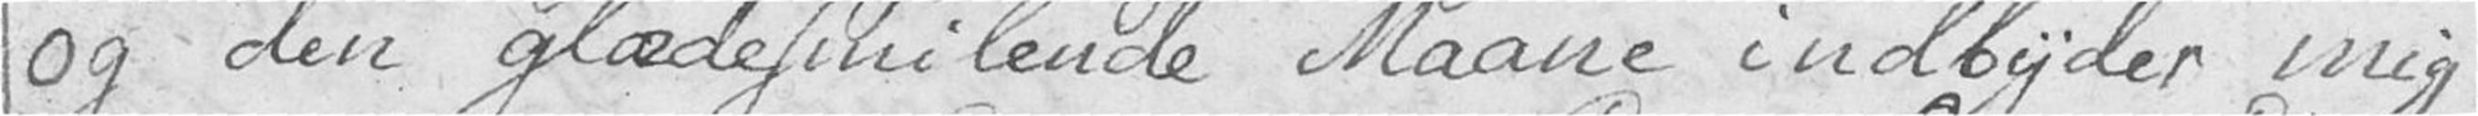og den glædesmilende Maane indbyder mig
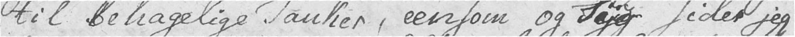til behagelige Tanker, eensom og Syg sider jeg
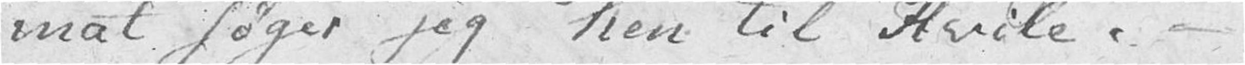mat søger jeg hen til Hvile. –
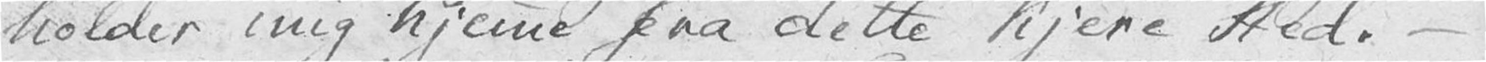holder mig hjemme fra dette kjere Sted. –
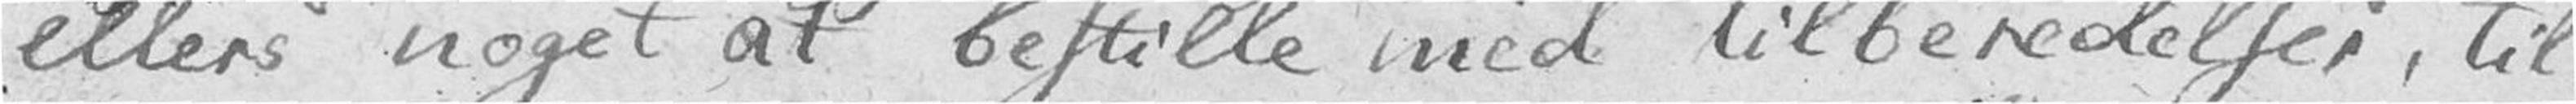ellers noget at bestille med tilberedelser, til
